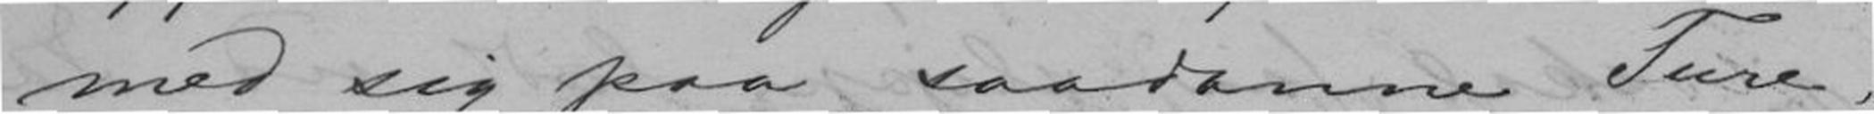med sig paa saadanne Ture,
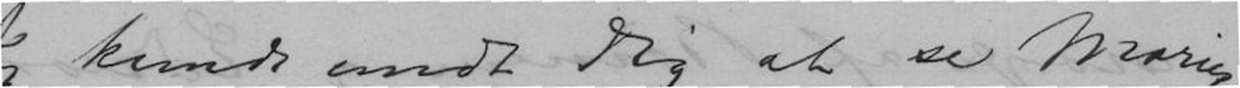Jeg kunde undt Dig at se Marie
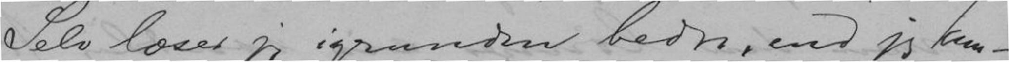Selv læser jeg igrunden bedre, end jeg kun-
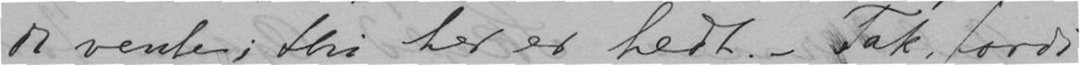de vente; thi her er hedt. - Tak, fordi
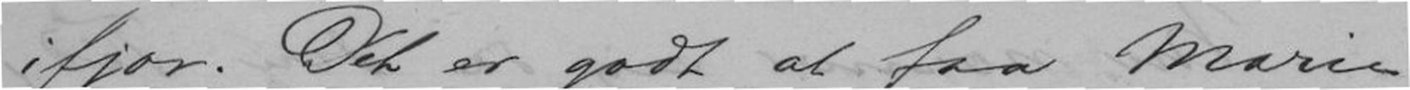ifjor. Det er godt at faa Marie
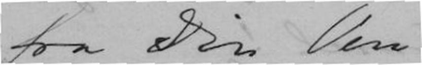fra Din Ven
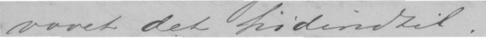vovet det hidindtil.
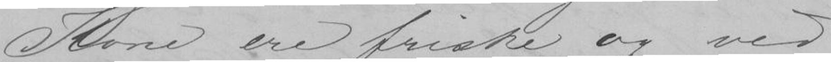Kone ere friske og ved
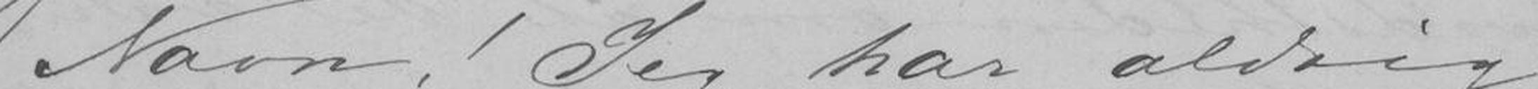Navn! Jeg har aldrig
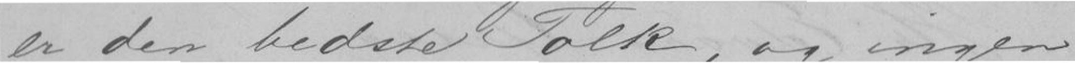er den bedste Tolk, og ingen
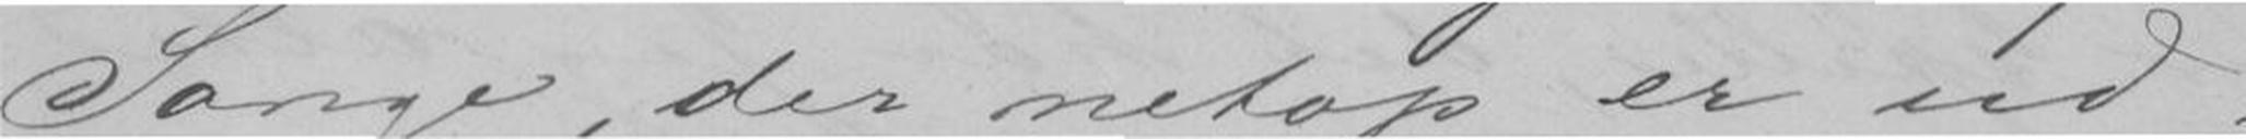Sange, der netop er ud-
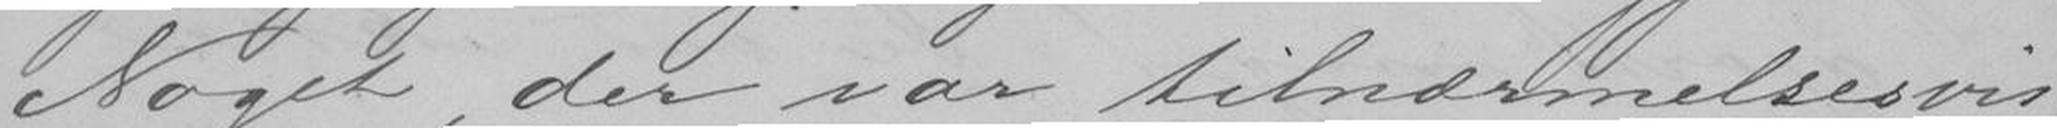Noget, der var tilnærmelsesvis
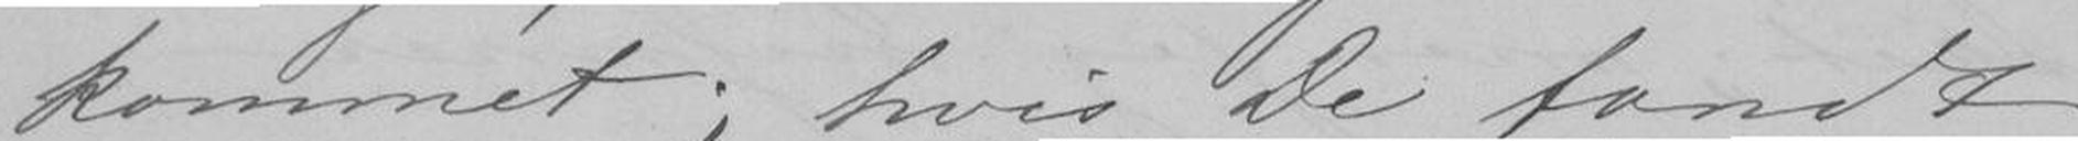kommet; hvis De fandt
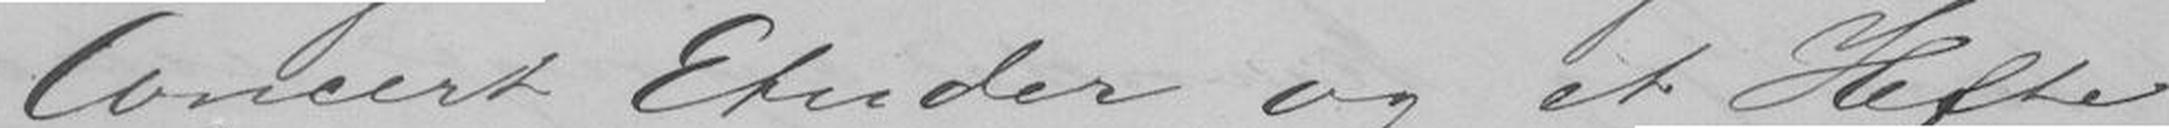Concert Etuder og et Hefte
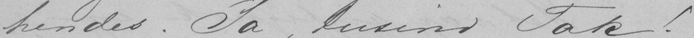hendes. Ja, tusind Tak!
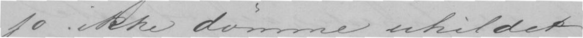jo ikke dømme uhildet.
